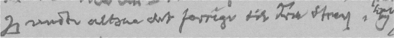Jeg sendte altsaa det forrige til Fru Stray, og
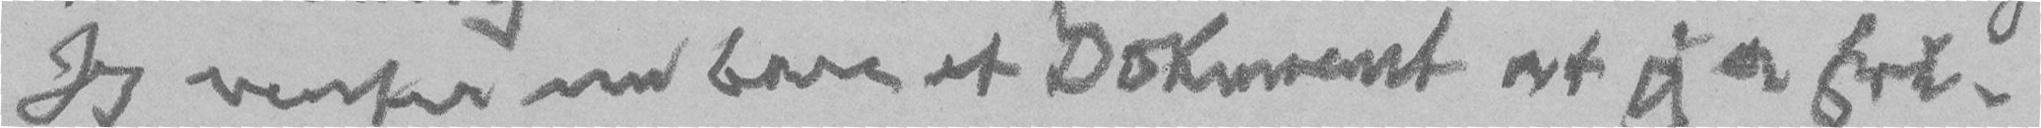Jeg venter nu bare et Dokument at jeg er fri.
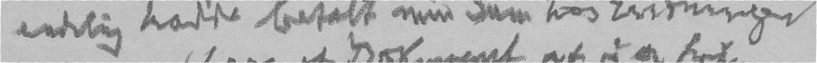endelig hadde betalt min Sum hos Erstningen
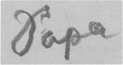Papa
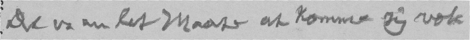Det var en let Maate at komme sig væk
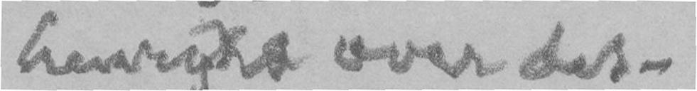henrykt over det.
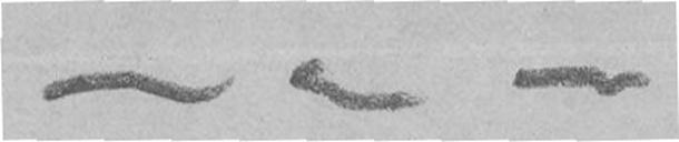- - -
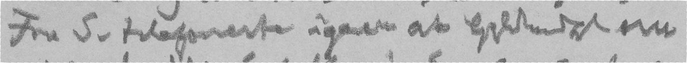Fru S. telefonerte igaar at Gyldendal nu
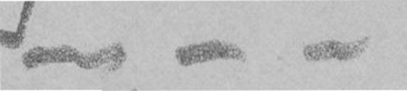- - -
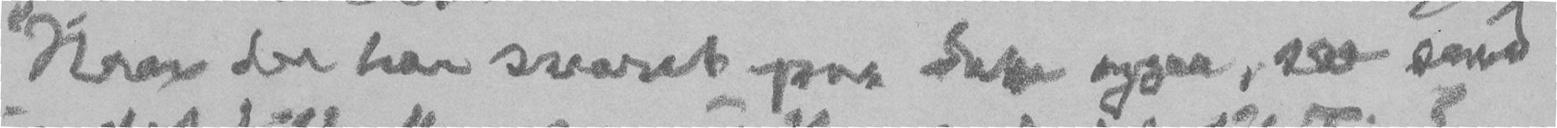Naar du har svaret paa dette ogsaa, saa send
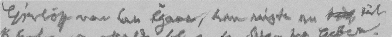Gierløff var her igaar, han ringte nu til til
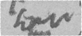hun
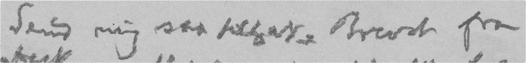Send mig saa tilbake Brevet fra
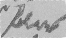blev
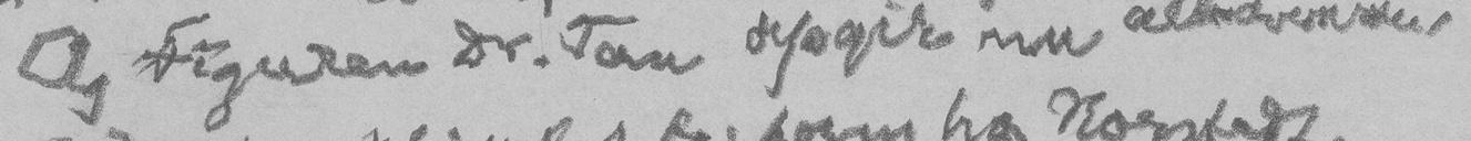Og Figuren Dr. Tau opgir nu allesammen,
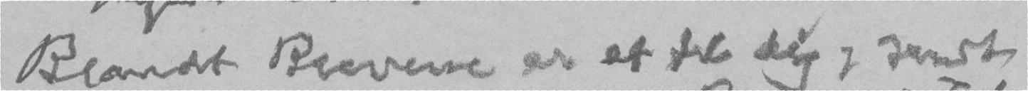Blandt Brevene er et til dig, sendt
